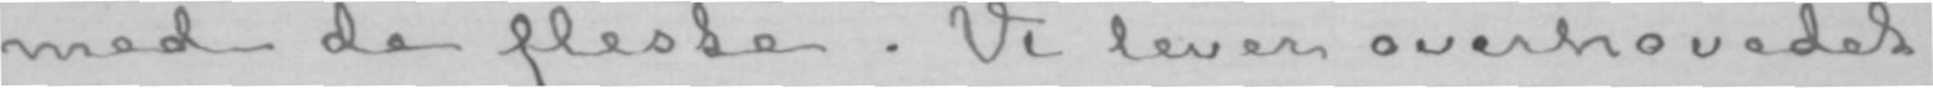med de fleste. Vi lever overhovedet
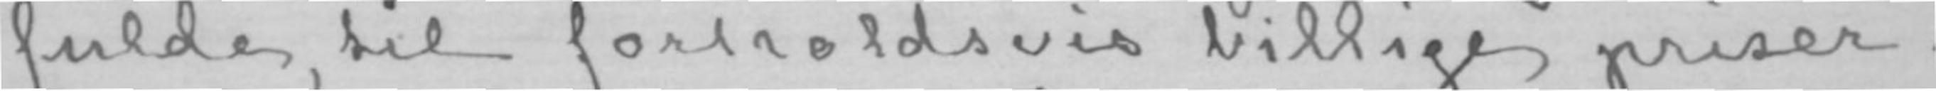fulde, til forholdsvis billige priser.
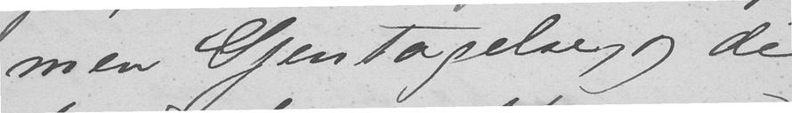men Gjentagelse, og de
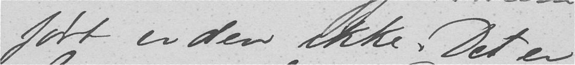ført er den ikke. Det er
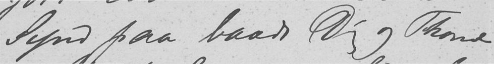Synd paa baade Dig og Thorne
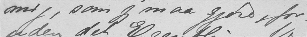mig, som jeg maa gjøre for-
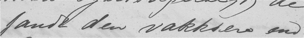fandt den vakkrere end
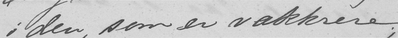i den, som er vakkrere,
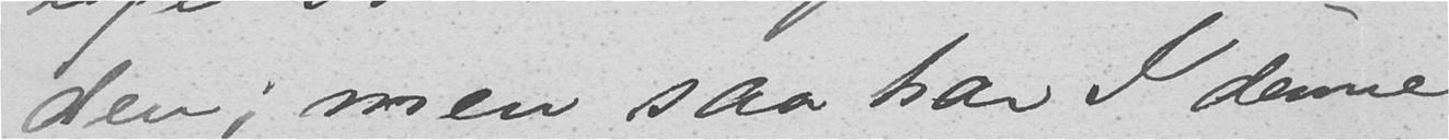den, men saa har I denne
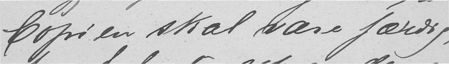Copien skal være færdig,
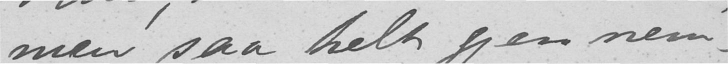men saa helt gjennem-
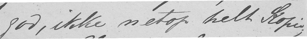god, ikke netop helt Kopie,
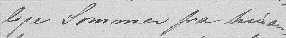lige Sommer fra hinan-
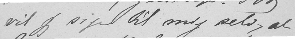vil jeg sige til mig selv, at
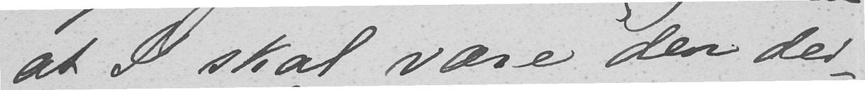at I skal være den dei-
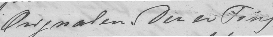Orignalen. Der er Ting
